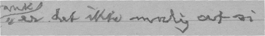"er det ikke mulig at si
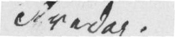Fredag.
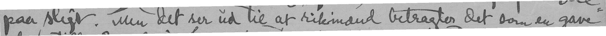paa sligt. Men det ser ud til at rikmand betragter det som "en gave"
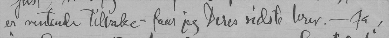er ventende tilbake - faar jeg Deres sidste brev - Ja,
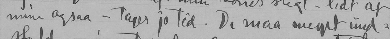mine ogsaa - tager jo tid. De maa meget und-
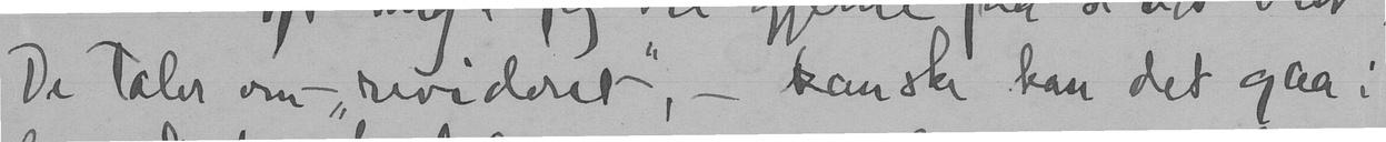De taler om - "revideret" - kanske kan det gaa i
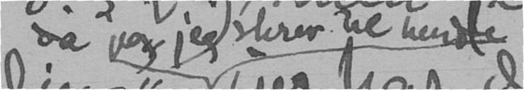da jeg jeg skrev til hende
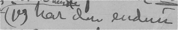- (jeg har den endnu
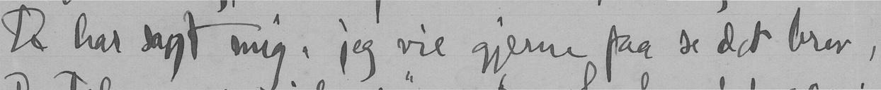De har sagt mig, jeg vil gjerne faa si det brev,
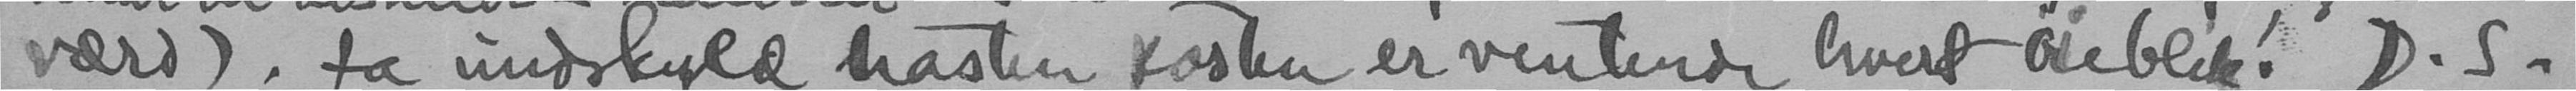værd). Ja undskyld hasten posten er ventende hvert øieblik! D.S.
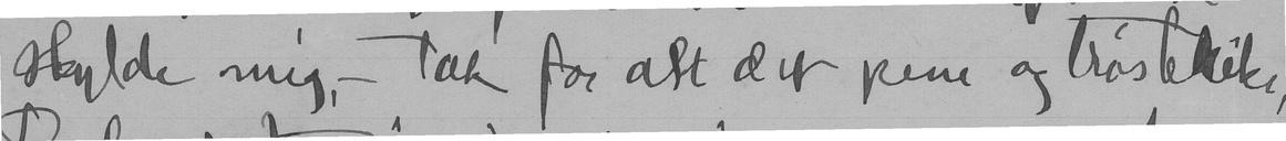skylde mig, - tak for alt det pene og trøstelike,
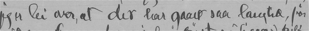jeg er lei over, at det har gaaet saa langtid, før
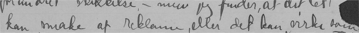kan smake af reklame, eller det kan virke som
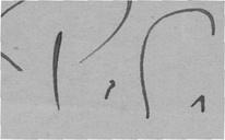P.f.
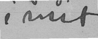i mit
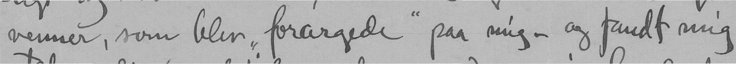venner, som blev "forargede" paa mig,- og fandt mig
